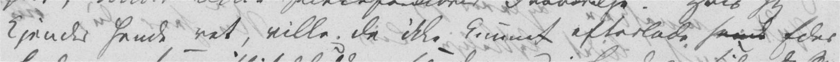kjender hende ret, ville de ikke kunnet efterlade hende Eder
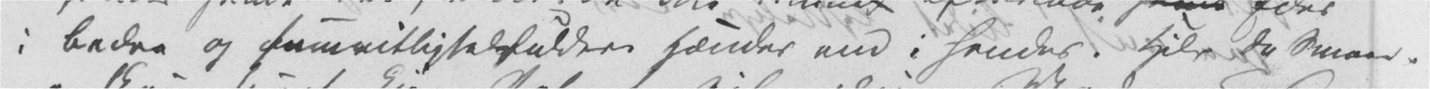i bedre og samvittighedsfuldere Hænder end i hendes. Hils de Smaae,
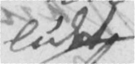lidet
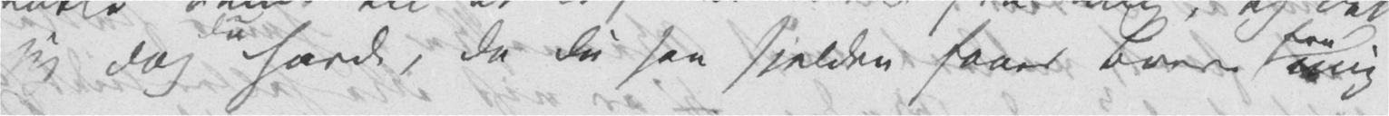jeg dog Du havde, da Du saa sjelden faaer Breve mig.
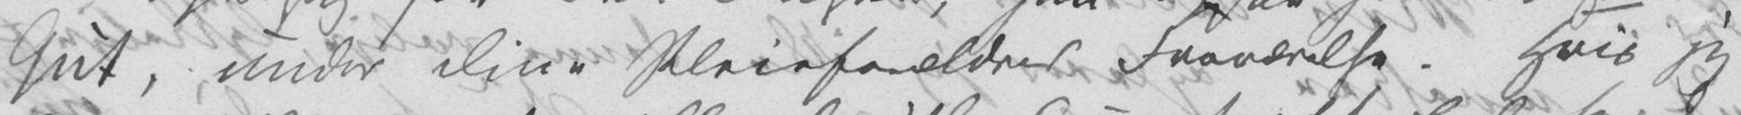Gut, under Dine Pleieforældres Fraværelse. Hvis jeg
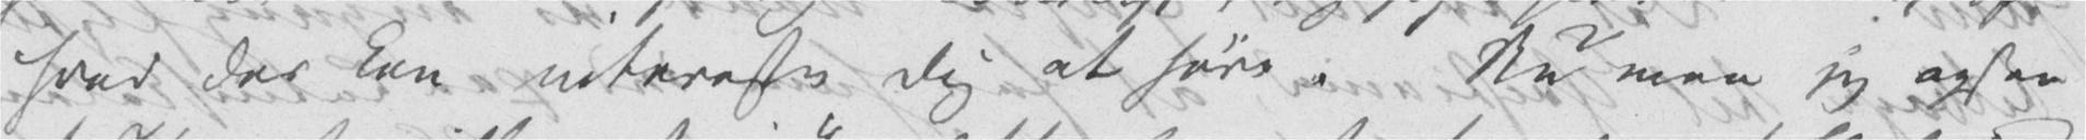hvad der kan interessere Dig at høre. Nu maa jeg ogsaa
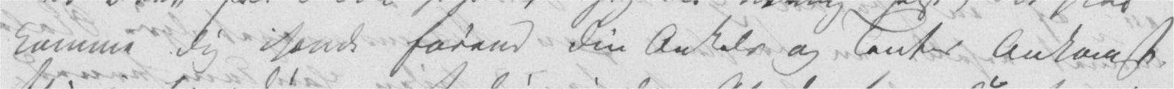komme Dig ihænde førend Din Onkels og Tantes Ankomst,
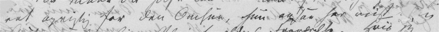ret oprigtig for den Omhu, hun ogsaa har viist min
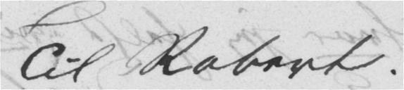Til Robert.
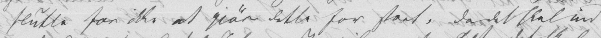slutte for ikke at gjøre dette for stort, da det skal ind
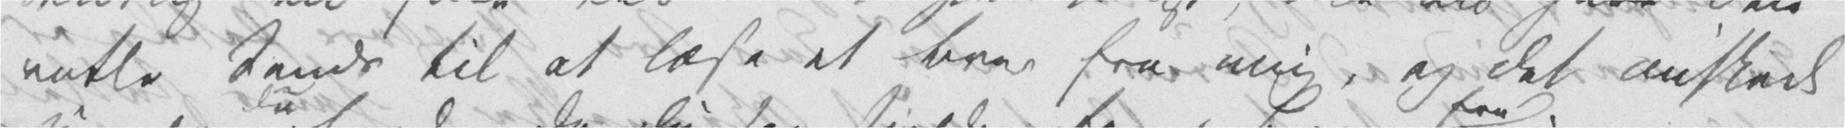rette Sands til at læse et Brev fra mig, og det ønskede
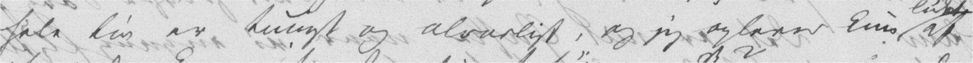hele Liv er tungt og alvorligt, og jeg oplever kun af
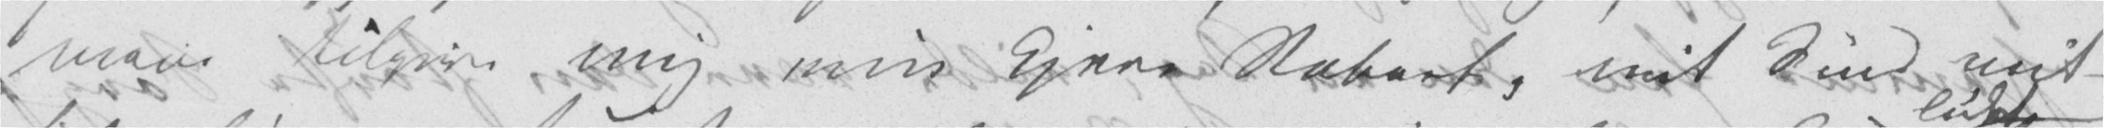maae tilgive mig min kjere Robert, mit Sind mit
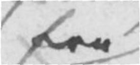fra
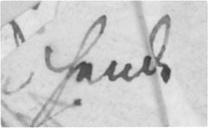hende
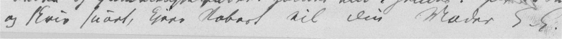og skriv snart, kjere Robert til Din Moder C. C.
